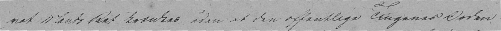vat A lands Ret krænkes uden at den offentlige Tingenes Orden
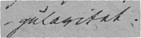gularitet.
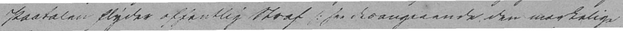Paatalen flyder offentlig Straf /: see desangaaende den mærkelige
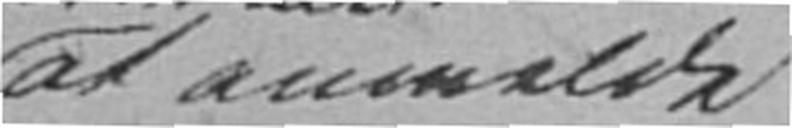at anmelde
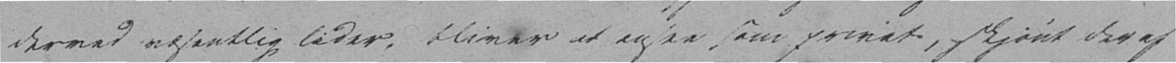derved væsentlig lider, bliver at ansee som privat, skjønt der af
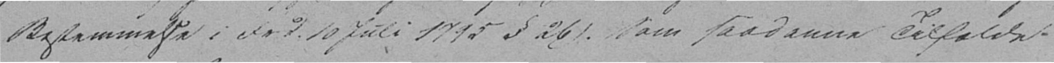Bestemmelse i Frd. 10 Juli 1795 § 261. som saadanne Tilfælde
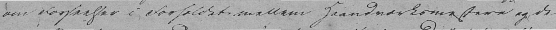om Forseelser i Forholdet mellem Haandværksmestere og de-
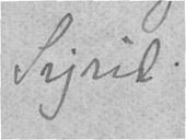Sigrid.
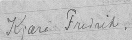Kjære Fredrik,
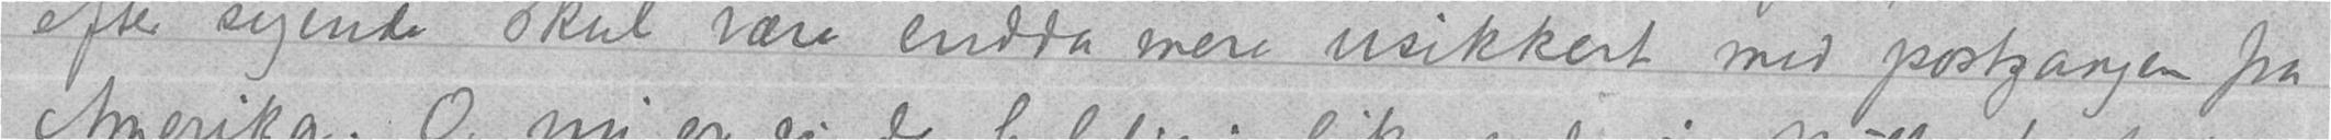efter sigende skal være endda mere usikkert med postgangen fra
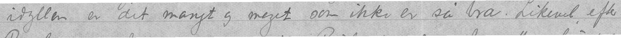idyllen er det mangt og meget som ikke er så bra. Likevel, efter
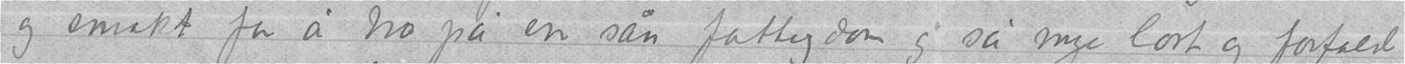og smakt for å tro på en sån fattigdom og så mye lort og forfald
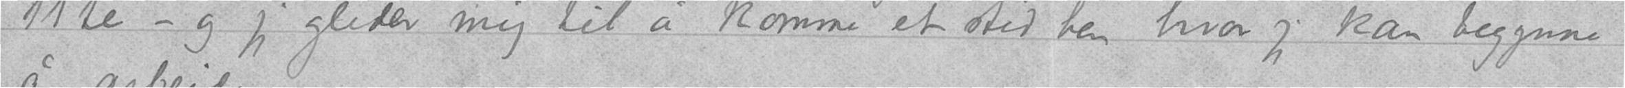11te – og jeg gleder mig til å komme et sted hen hvor jeg kan begynne
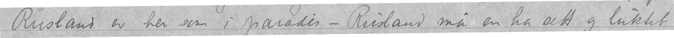Rusland er her som i paradis - Rusland må en ha sett og luktet
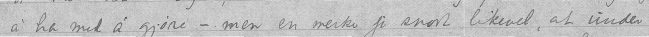å ha med å gjøre – men en merker jo snart likevel, at under
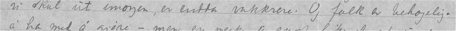vi skal ut imorgen, er endda vakkrere. Og folk er behagelige
